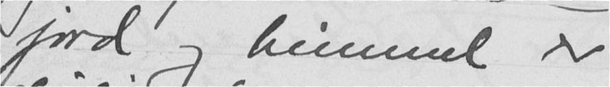jord og himmel er
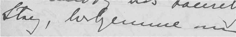Stang, herhjemme om
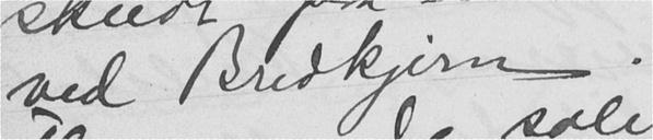ved Bredkjern.
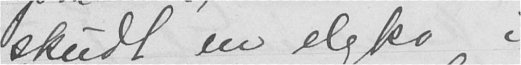skudt en elgko i
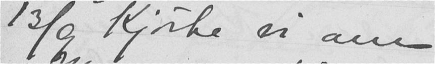13/9 Kjørte vi om
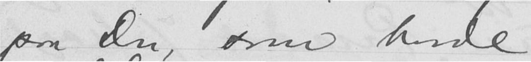paa Don, som havde
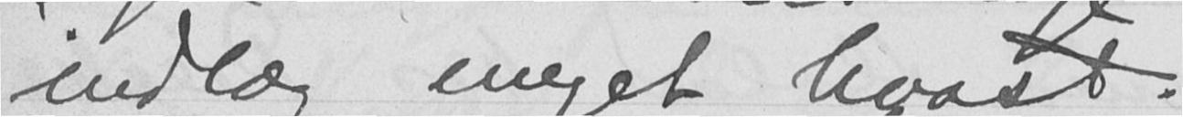indlæg meget hvast.
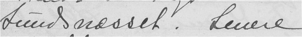Sundsnæsset. Senere
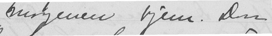morgenen hjem. Don
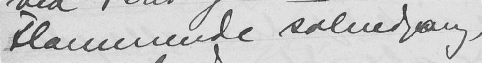Flammende solnedgang
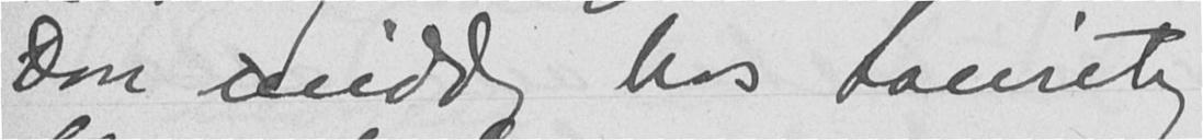Don middag hos Lauritz
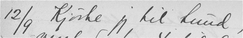12/9 Kjørte jeg til Sund,
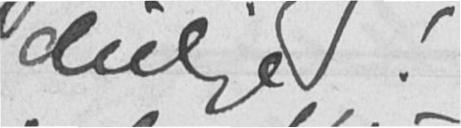deilige!
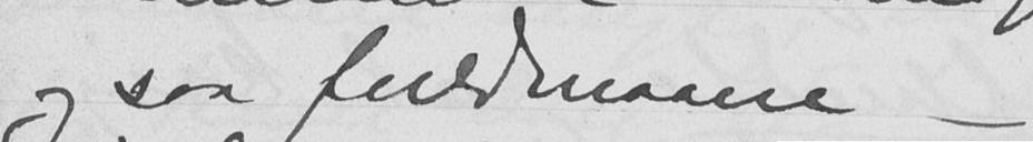og saa fuldmaane -
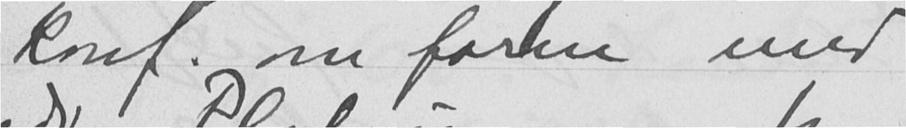konf. om form med
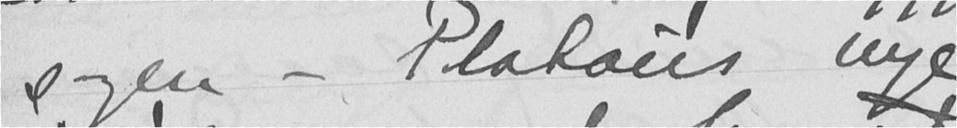sagen - Platous nye
